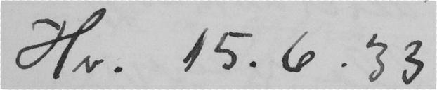Hv. 15.6.33
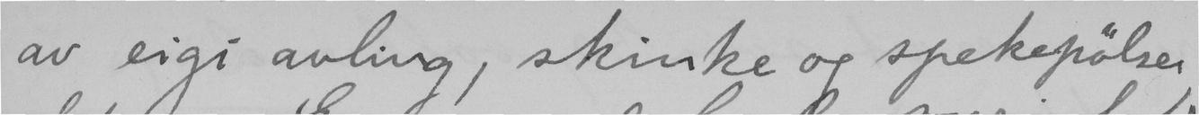av eigi avling, skinke og spekepölser
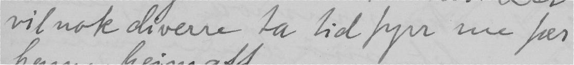vil nok diverre ta tid fyrr me fær
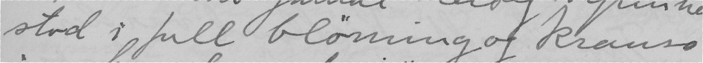stod i full blöming og kranso
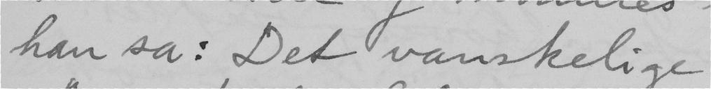han sa: Det vanskelige
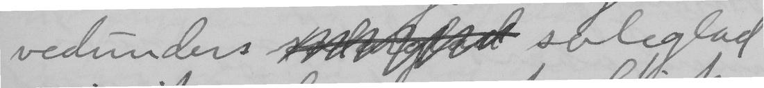vedunders  soleglad
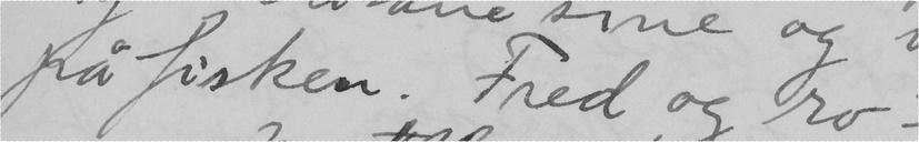på fisken. Fred og ro –
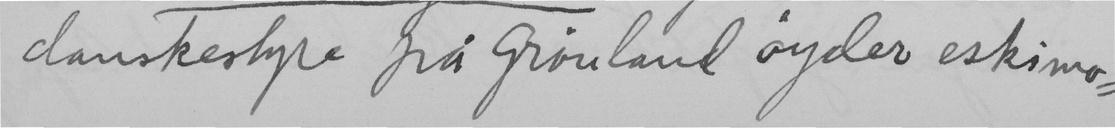danskestyre på Grönland öyder eskimo-
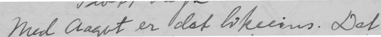Med Aagot er det likeeins. Det
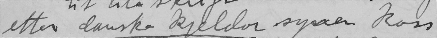etter danske kjeldor syner koss
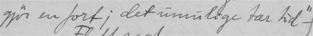gjör en fort; det umulige tar tid –
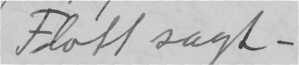Flott –
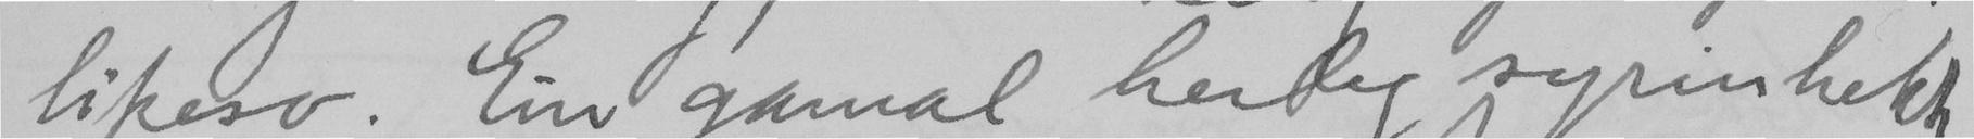likeso. Ein gamal herleg syrinhekk
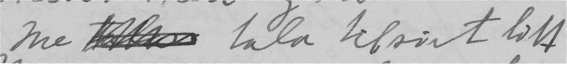Me  tala tilsist litt
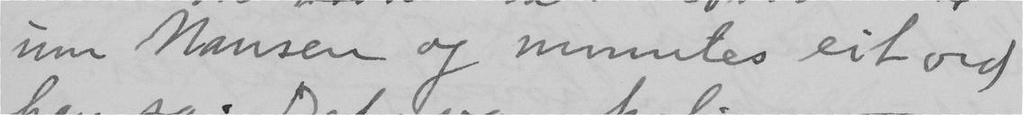um Nansen og mintes eit ord
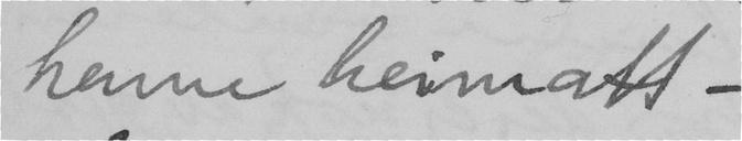henne heimatt –
